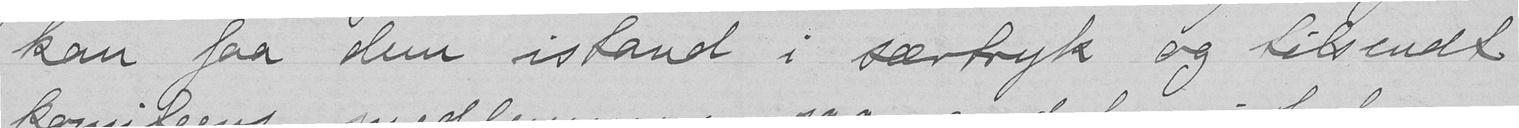kan faa dem istand i særtryk og tilsendt
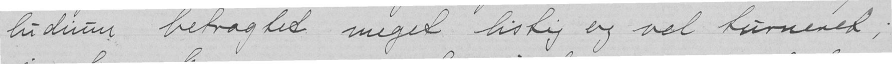ludim betragtes meget listig og vel turneret;
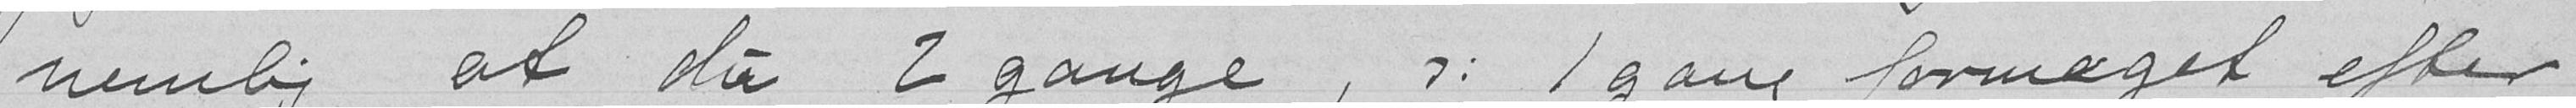nemlig at du 2 gange, : 1gang formæget efter
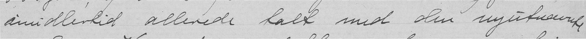imidlertid allerede talt med den nyutnevnte
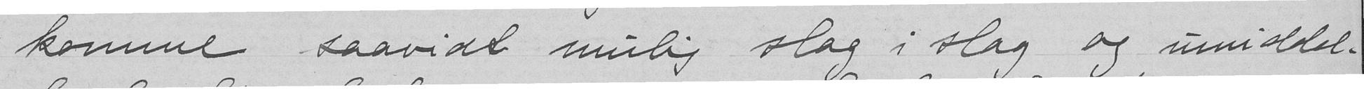komme saavidt mulig slag i slag og umiddel-
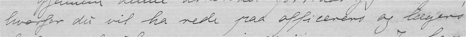hvorfor der vil fa rede paa officerers og legers
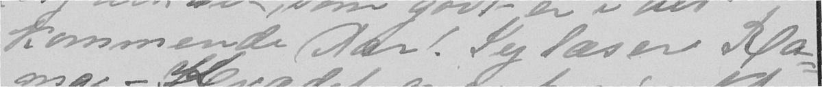kommende Aar! Jeg læser Ra-
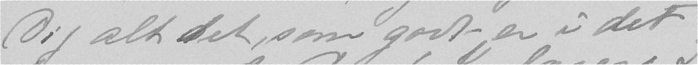Dig alt det, som godt er i det
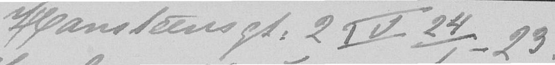Hansteensgt. 2 IV 24/1-23.
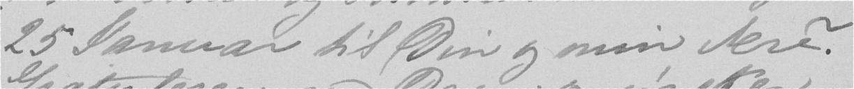25 Januar til Din og min Ære?
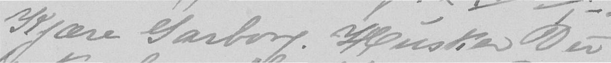Kjære Garborg. Husker Du
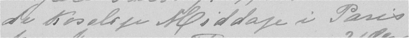de koselige Middage i Paris
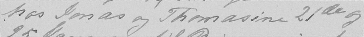hos Jonas og Thomasine 21de og
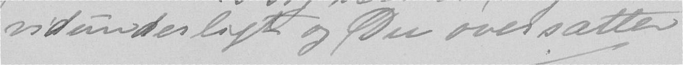vidunderligt og Du oversætter
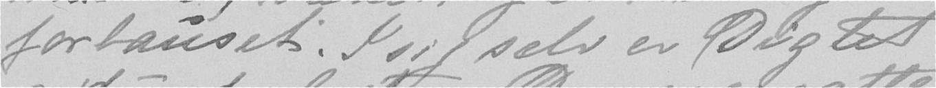forbauset. I sig selv er Digtet
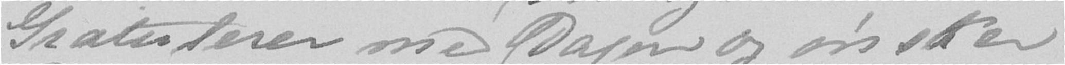Gratulerer med Dagen og ønsker
